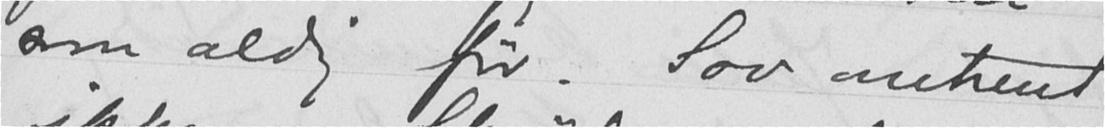som aldrig før. Sov omtrent
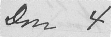Don 4.
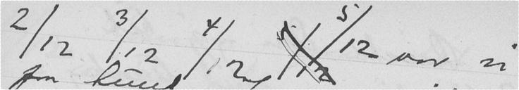2/12 3/12 4/12 5/12 5/12 var vi
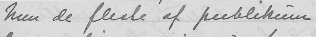Men de fleste af publikum
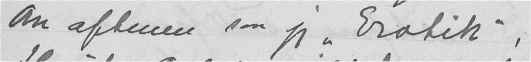Om aftenen saa jeg "Erotik",
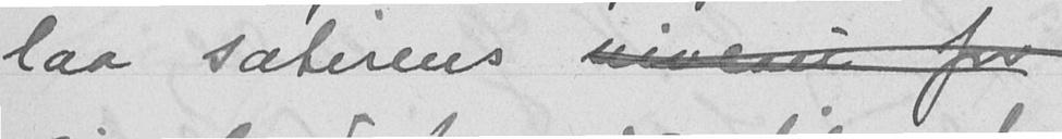laa satirens viveri for
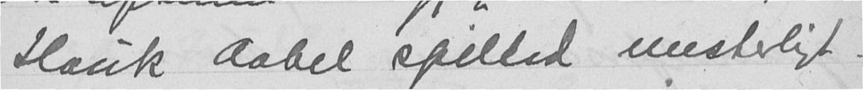Hauk Aabel spilled mesterligt.
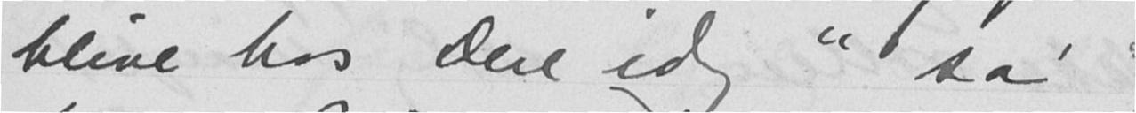blive hos Dere idag" sa'
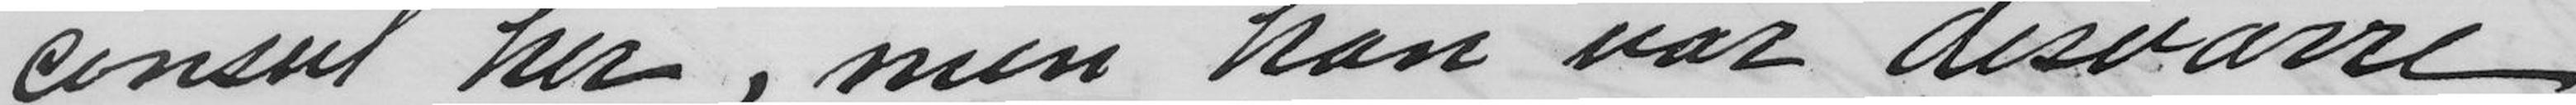consul her, men han var desværre
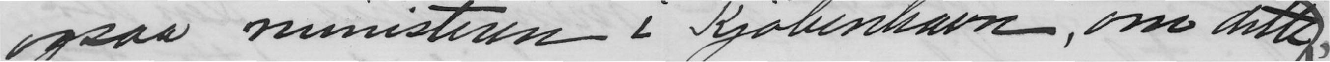ogsaa ministeren i Kjøbenhavn, om dette
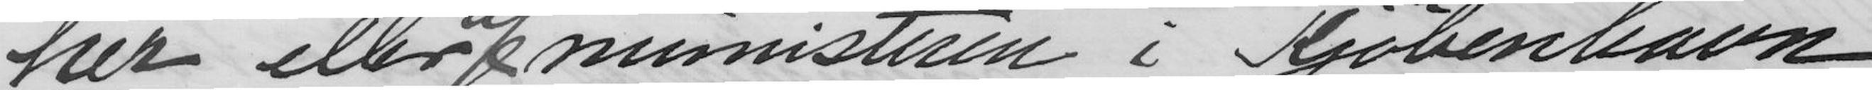her eller af ministeren i Kjøbenhavn
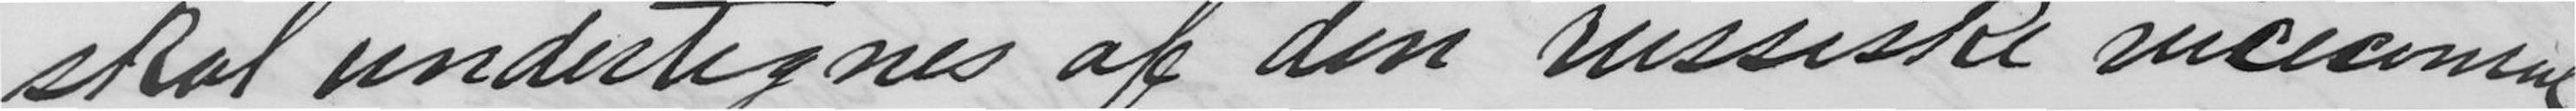skal undertegnes af den russiske viceconsul
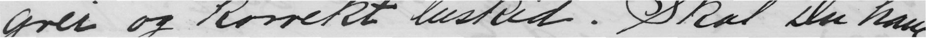grei og korrekt besked. Skal Du have
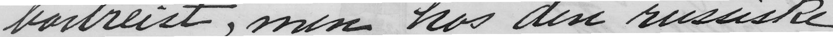bortreist, men hos den russiske
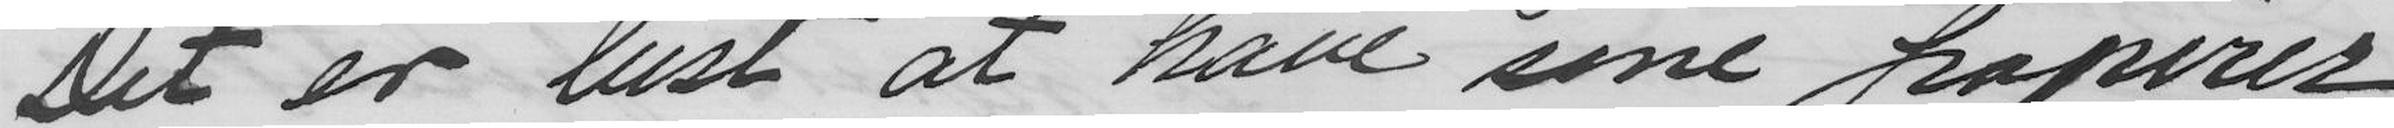Det er best at have sine papirer
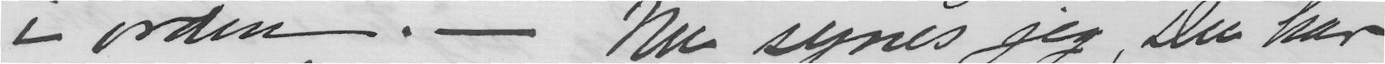i orden. - Nu synes jeg, Du har
